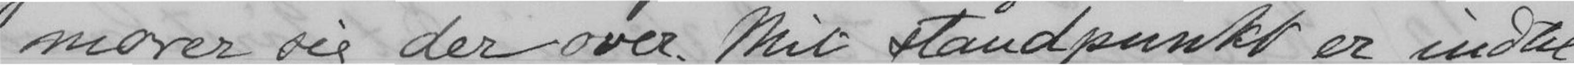morer sig der over. Mit standpunkt er indtil
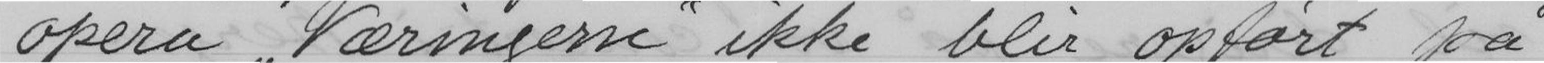opera Væringerne ikke blir opført på
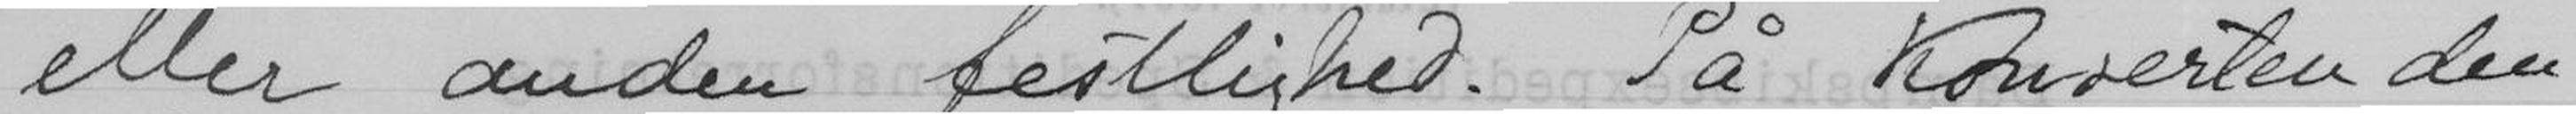eller anden festlighed. På konserten den
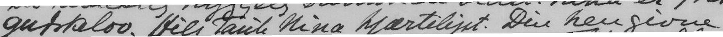gudskelov. Hils Tante Nina hjærteligst. Din hengivne
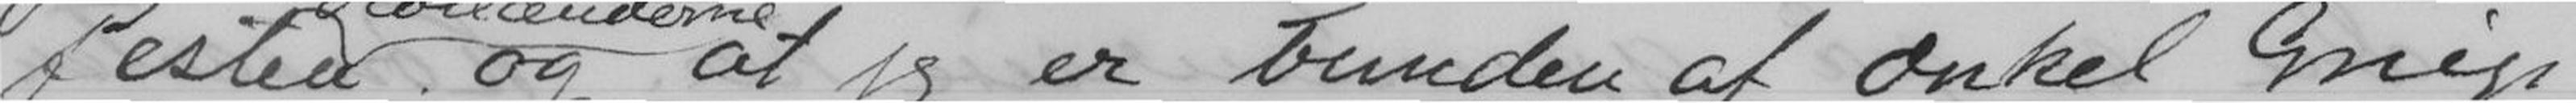festen at jeg er bunden af onkel Griegs
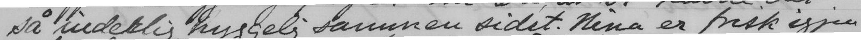så inderlig hyggelig sammen sidst. Nina er frisk igjen
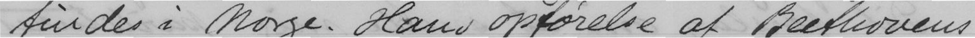findes i Norge. Hans opførelse af Beethovens
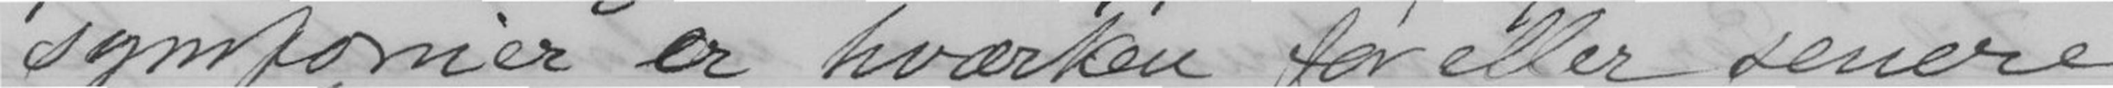symfonier er hværken før eller senere
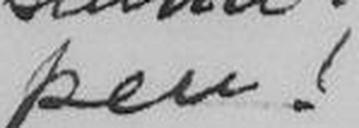pen!
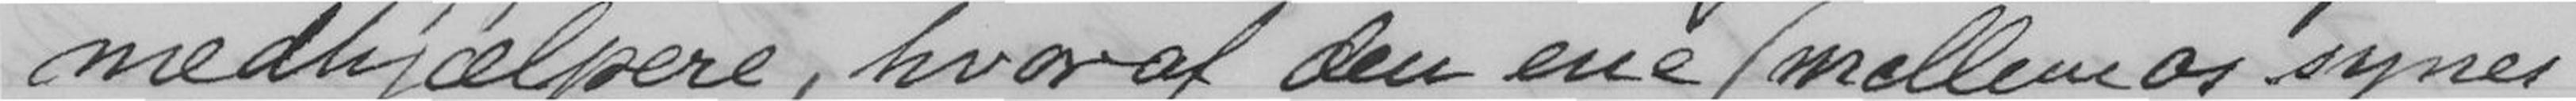medhjælpere, hvoraf den ene (mellem os synes
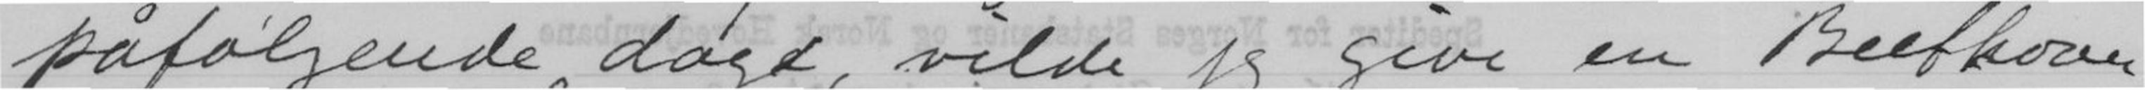påfølgende dag, vilde jeg give en Beethoven
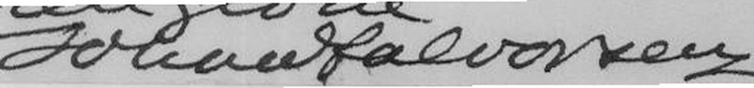Johan Halvorsen
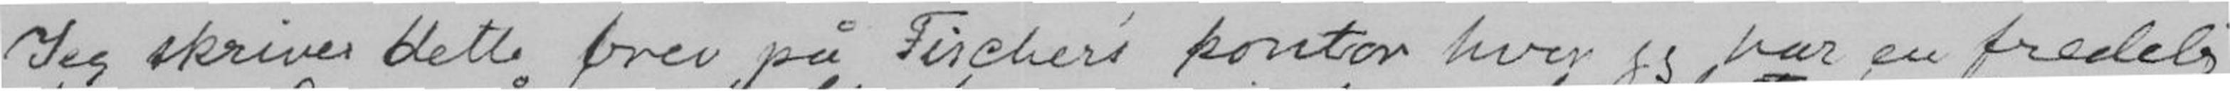Jeg skriver dette brev på Fischers kontor hvor jeg har en fredelig
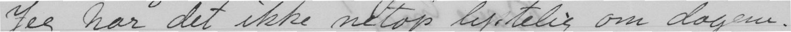Jeg har det ikke netop lystelig om dagene.
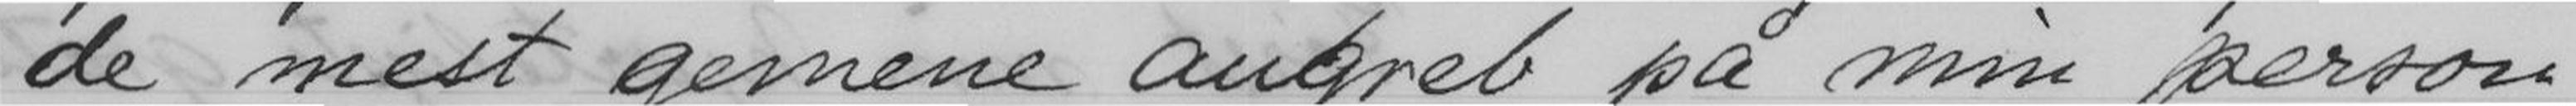de mest gemene angreb på min person
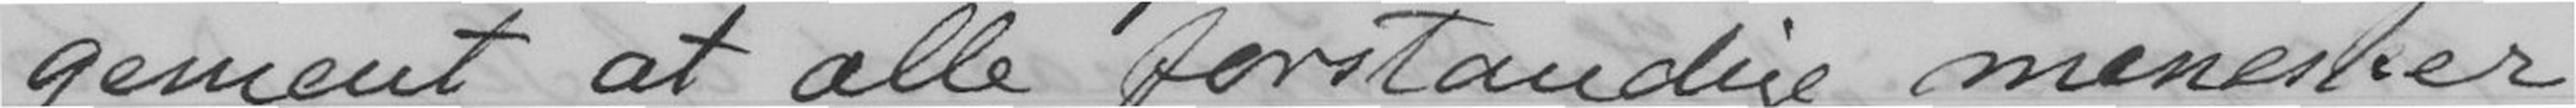gement at alle forstandige mennesker
